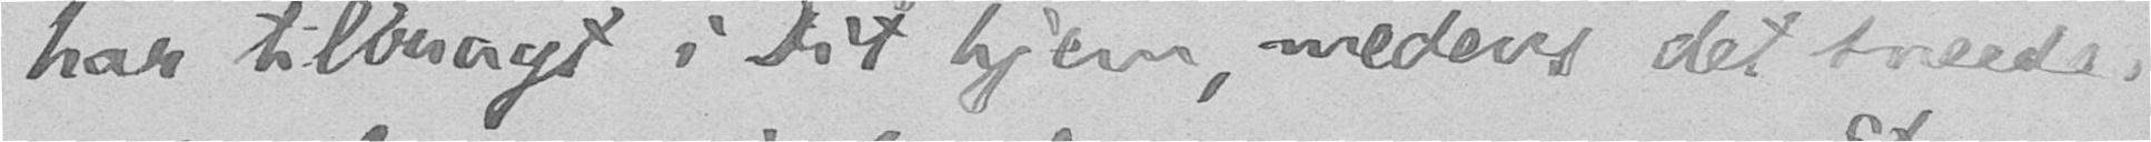har tilbragt i Dit hjem, medens det sneede
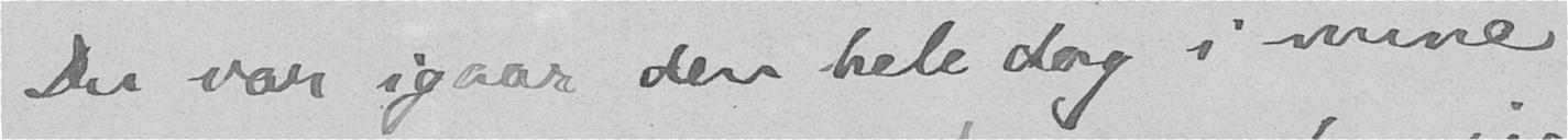Du var igaar den hele dag i mine
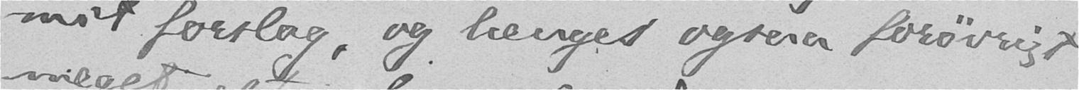mit forslag, og længes ogsaa forøvrigt
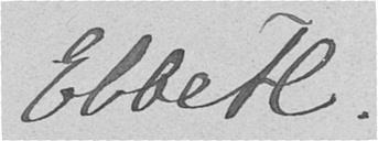Ebbe H.
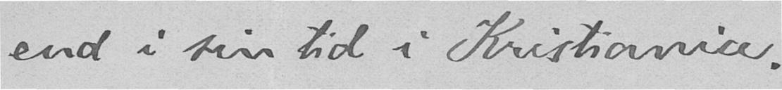end i sin tid i Kristiania.
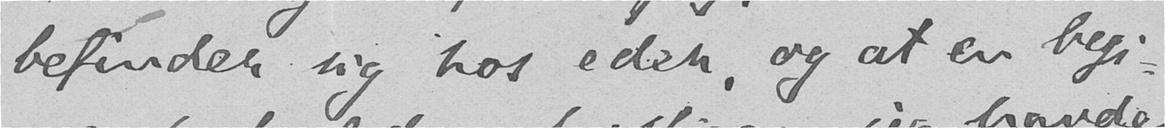befinder sig hos eder, og at en begi-
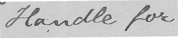Handle for
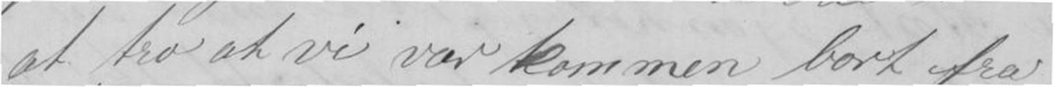at tro at vi var kommen bort fra
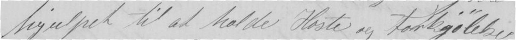hjulpet til at holde Hoste og Forkjølelse
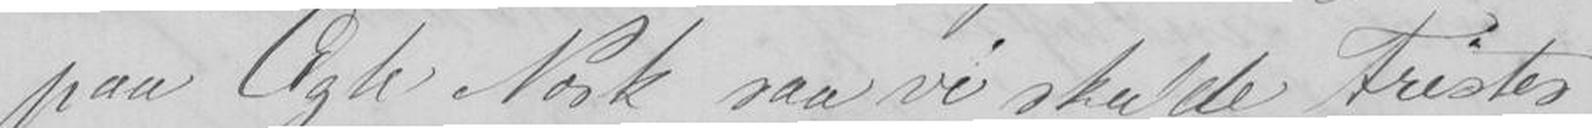pa Ægte Norsk saa vi skulde Fristes
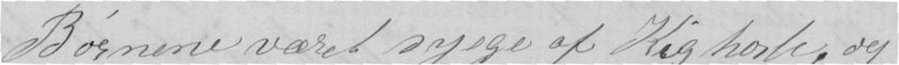Børnene været syge af Kighoste og
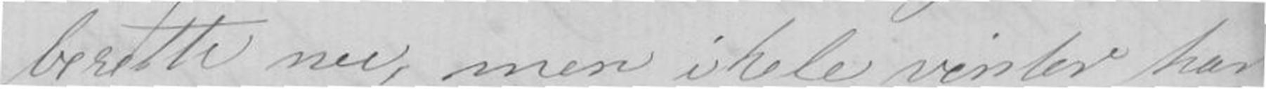berette nu, men ihele vinter har
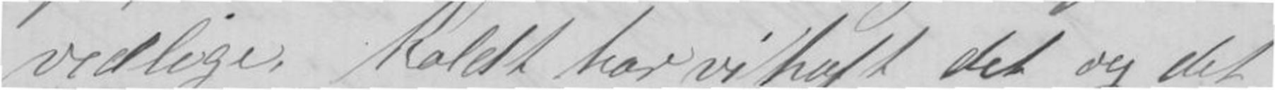vedlige. Koldt har vi haft det og det
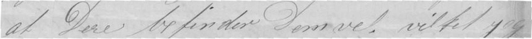at Dere befinder Dem vel, vilket jeg
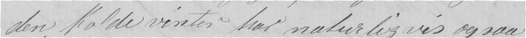den Kolde vinter har naturligvis ogsaa
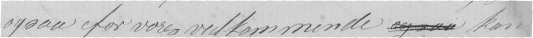ogsaa for vores vedkommende ogsaa kan
